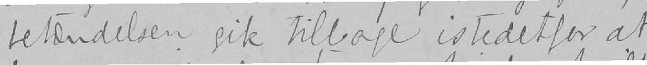betændelsen gik tilbage istedetfor at
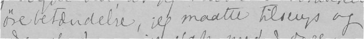ørebetændelse, jeg maatte tilsengs og
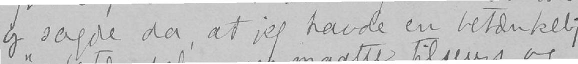og sagde da, at jeg havde en betænkelig
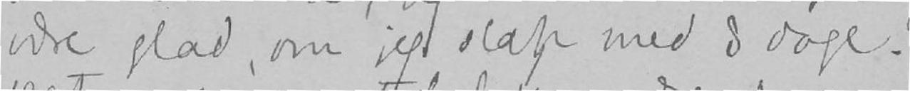være glad, om jeg slap med 8 dage.
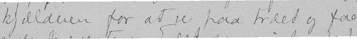kjælderen for at se paa træet og faa
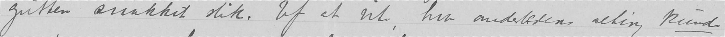gutten snakket slik. Uf at vite, hvor anderledens alting kunde
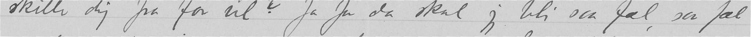skille dig fra far vel? Ja for da skal jeg bli saa fæl, saa fæl
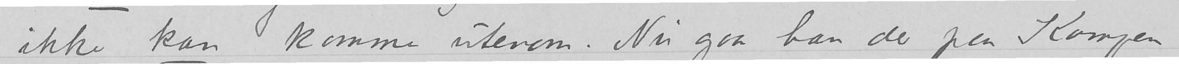ikke kan komme utenom. Nu gaar han der paa Kampen
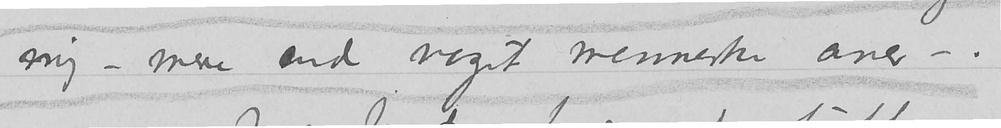mig – mere end noget menneske aner –
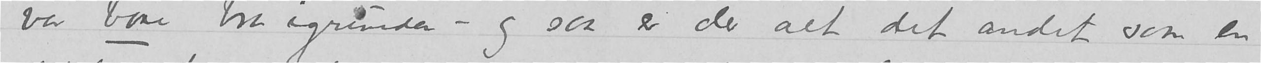var bare bra igrunden – og saa er der alt det andet som en
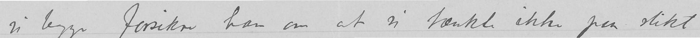vi begge forsikre ham om at vi tænkte ikke paa slikt
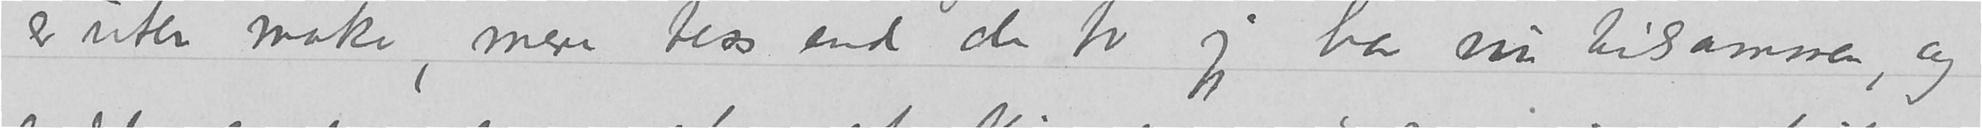er uten make, mere tess end de to jeg har nu tilsammen, og
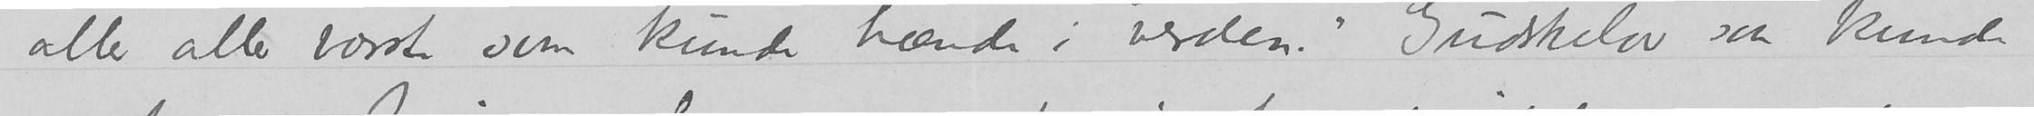aller aller værste som kunde hænde i verden.» Gudskelov saa kunde
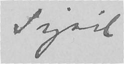Sigrid.
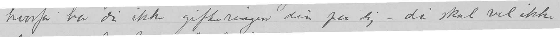hvorfor har du ikke gifteringen din paa dig - du skal vel ikke
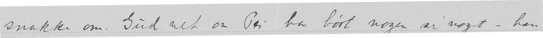snakke om. Gud vet om Pei har hørt nogen si noget – han
